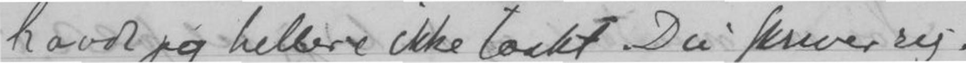havde jeg hellere ikke tænkt Du skriver rig-
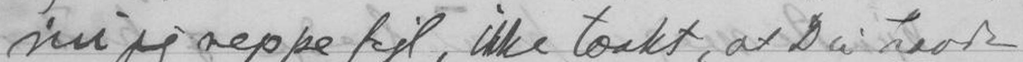nu jeg neppe fejl, ikke tænkt, at Du havde
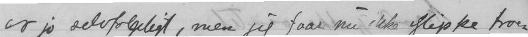er jo selvfølgeligt, men jeg faar nu ikke slippe troen
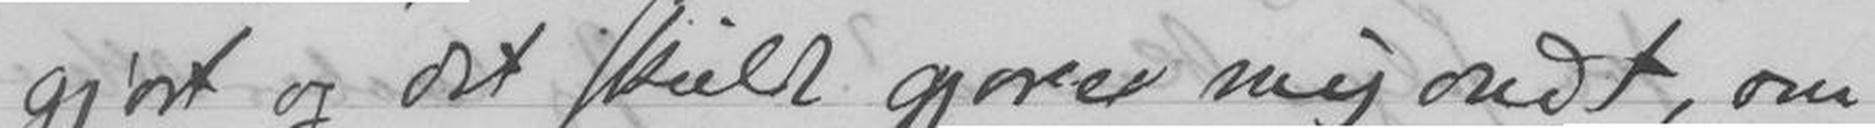gjort og det skulde gjøre mig ondt, om
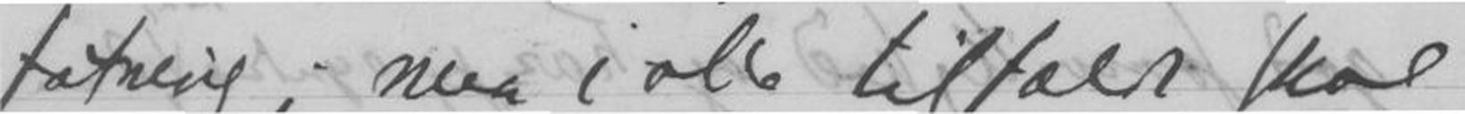fatning; men i alle tilfælde skal
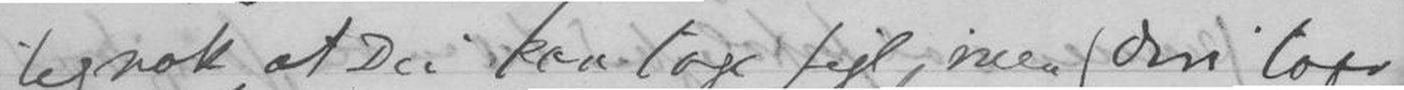tignok, at Du kan tage fejl, men (deri to.
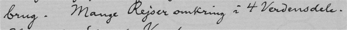brug. Mange Rejser omkring i 4 Verdensdele.
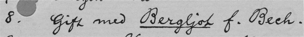8. Gift med Bergljot f. Bech.
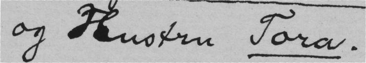og Hustru Tora.
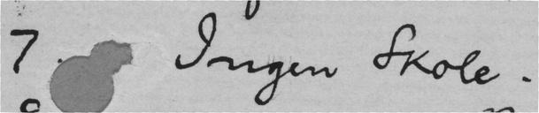7. Ingen Skole.
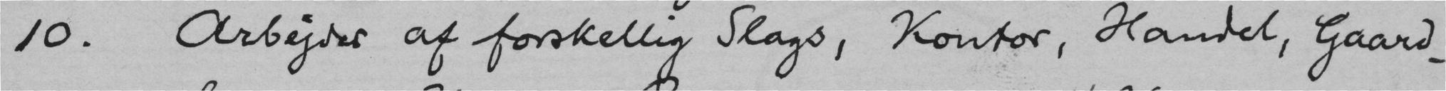10. Arbejder af forskellig Slags, Kontor, Handel, Gaard-
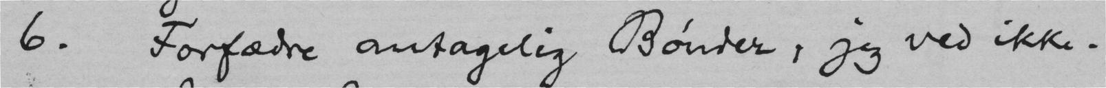6. Forfædre antagelig Bønder, jeg ved ikke.
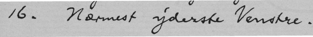16. Nærmest yderste Venstre.
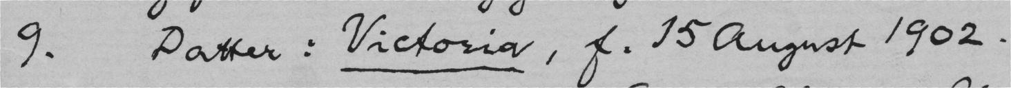9. Datter: Victoria, f. 15 August 1902.
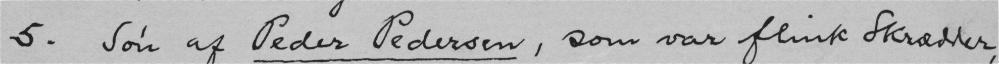5. Søn af Peder Pedersen, som var flink Skrædder,
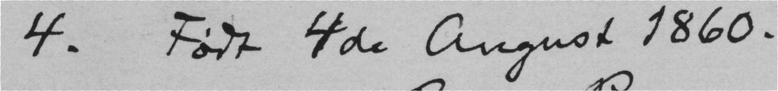4. Født 4de Angust 1860.
